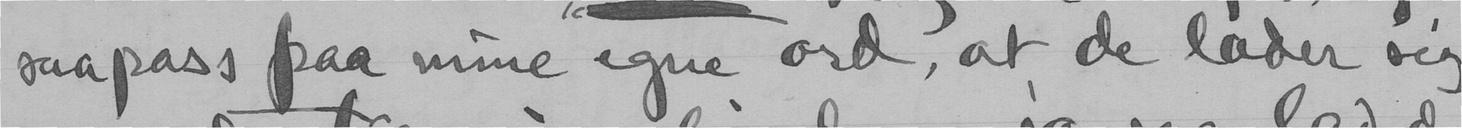saapass paa mine egne ord, at de lader sig
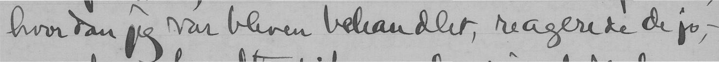hvordan jeg var bleven behandlet, reagerede de jo,-
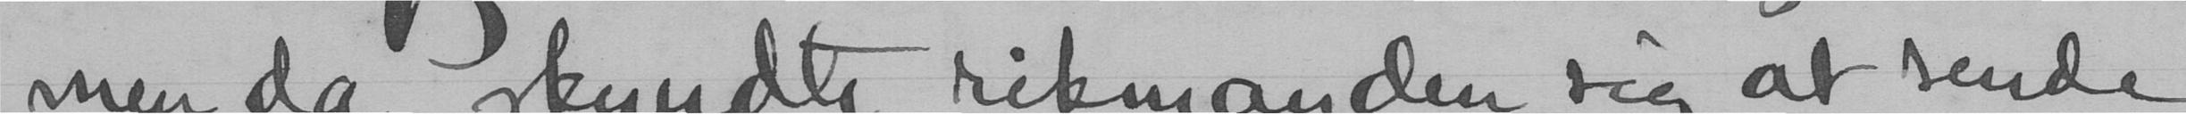men da skyndte rikmanden sig at sende
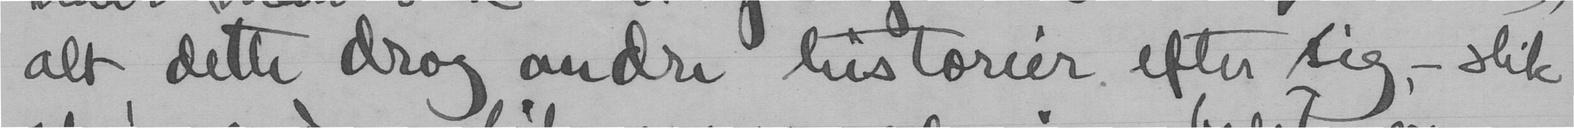alt dette drog andre historier efter sig, - slik
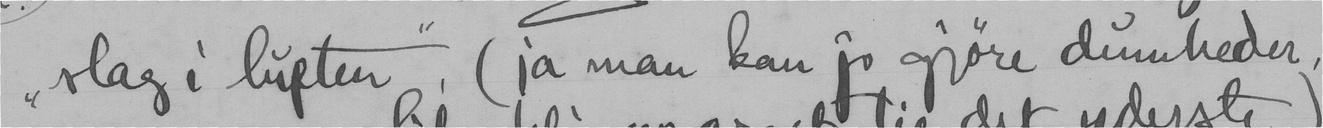"slag i luften", (ja man kan jo gjøre dumheder,
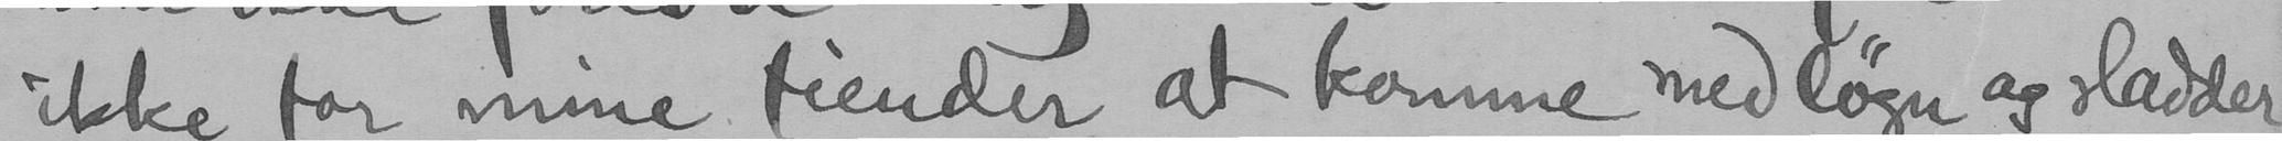ikke for mine fiender at komme med løgn op sladder.
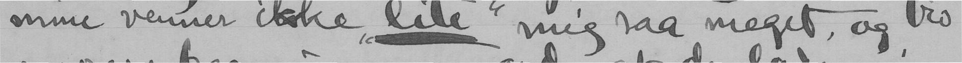mine venner ikke "lite" mig saa meget, og tro
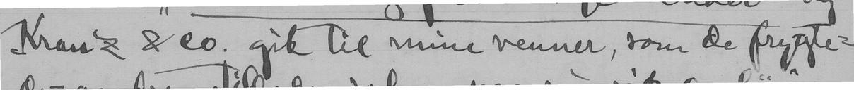Kranz & co. gik til mine venner, som de frygte-
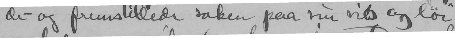de- og fremstillede saken paa sin vis og løi
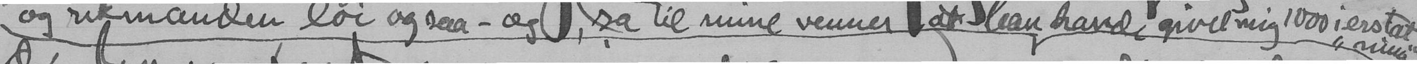og rikmanden løi ogsaa - og sa til mine venner at han havde givet mig 1000 i erstat-
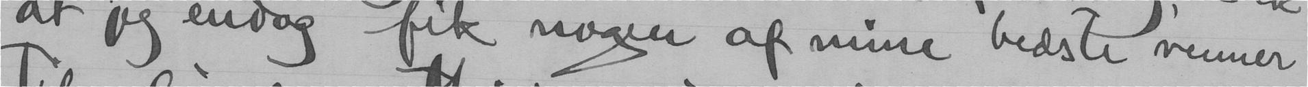at jeg endog fik nogen af mine bedste venner
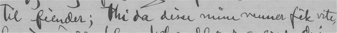til fiender: thi da disse mine venner fik vite,
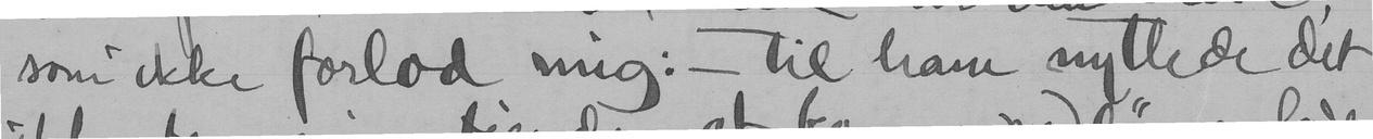som ikke forlod mig: - til ham nyttede det
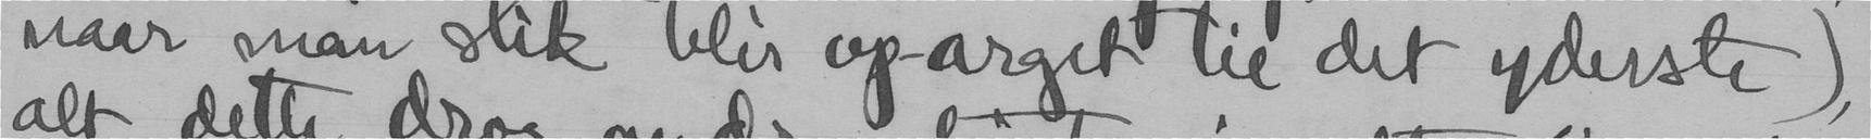naar man slik blir op-arget til det yderste),
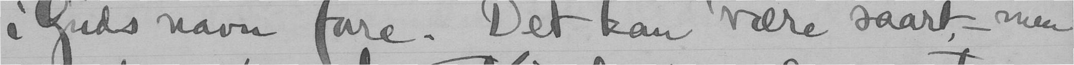i Guds navn fare. Det kan være saart, - men
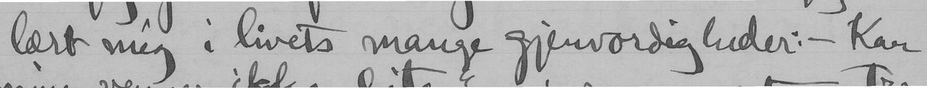lært mig i livets mange gjenvordigheder: - Kan
